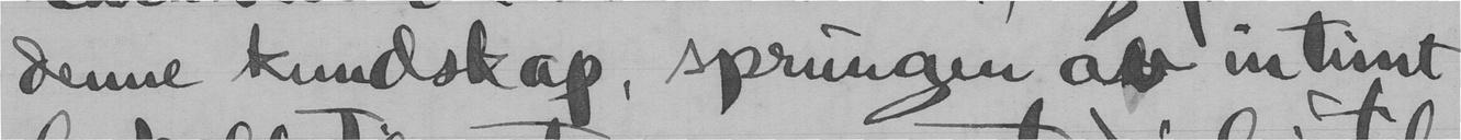denne kundskap, sprungen og intimt
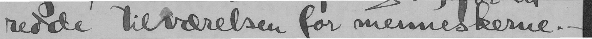redde tilværelsen for menneskerne.-
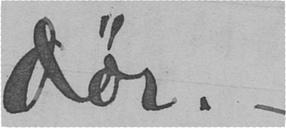dør. -
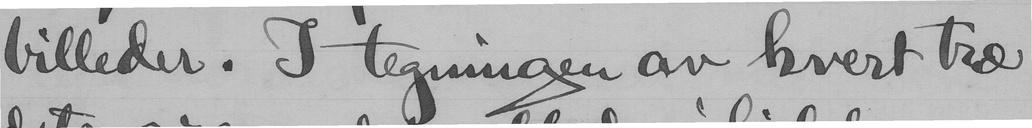billeder. I tegningen av hvert træ
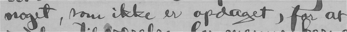noget, som ikke er opdaget, for at
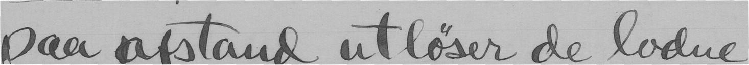paa afstand utløser de lodne
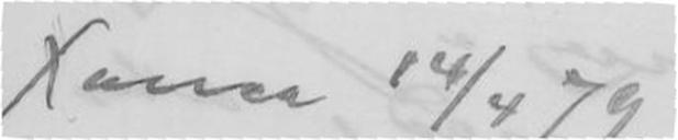Xania 14/4 79
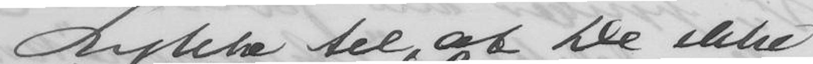Lykke til, at De ikke
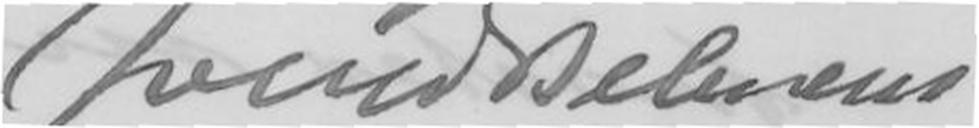Joh D Behrens
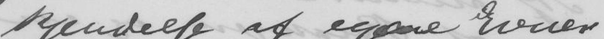kjendelse af egne Evner
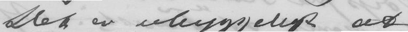Det er uhyggelig at
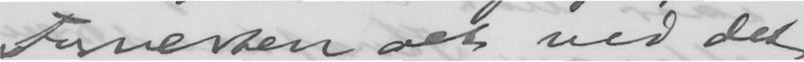Foresten alt ved det
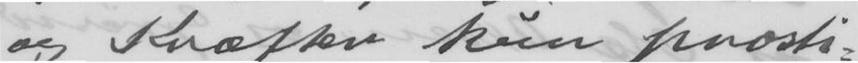og Kræfter kun prosti-
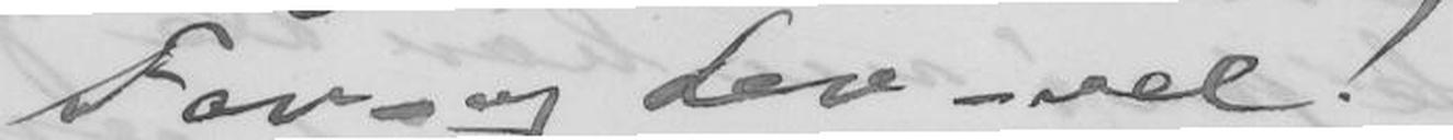Far- og lev-vel!
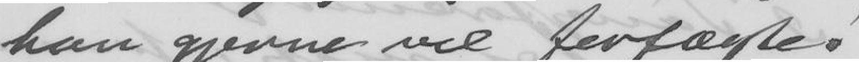han gjerne vil forfæstes
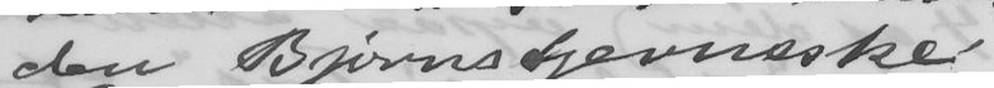den Bjørnstjerneske
